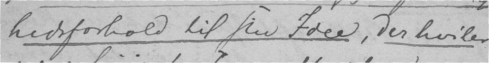hedsforhold til sin Idee, der hviler
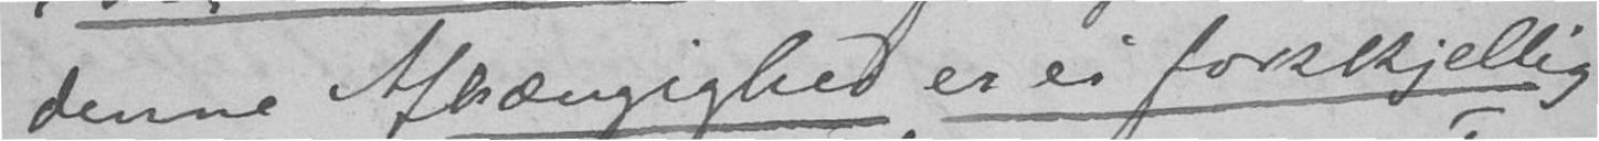denne Afhængighed er ei forskjellig
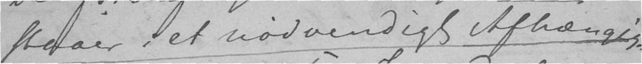staaer i et nødvendigt Afhængig-
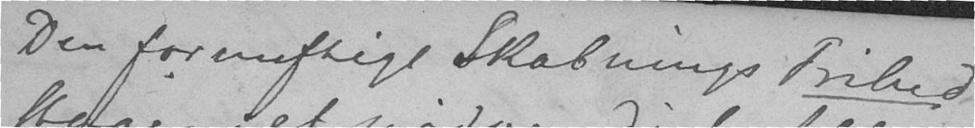Den fornuftige Skabnings Frihed
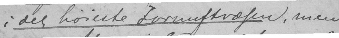i det høieste Fornuftvæsen, men
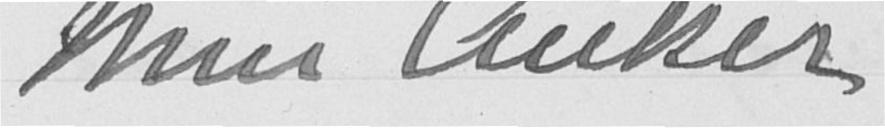Nini Anker
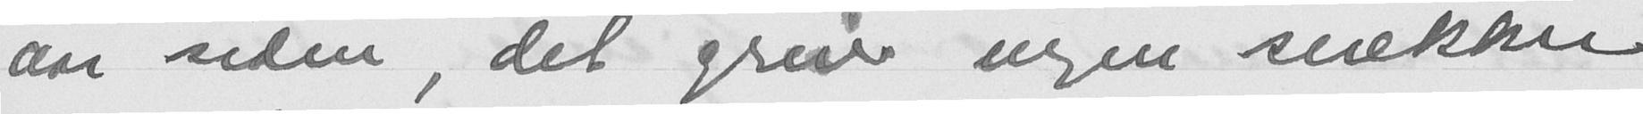aar siden, det greier ingen snekker
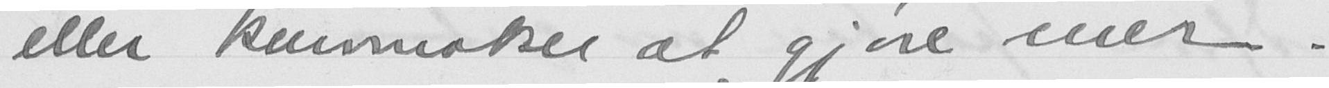eller kurvmaker at gjøre mer.
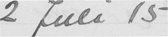2 Juli 1915
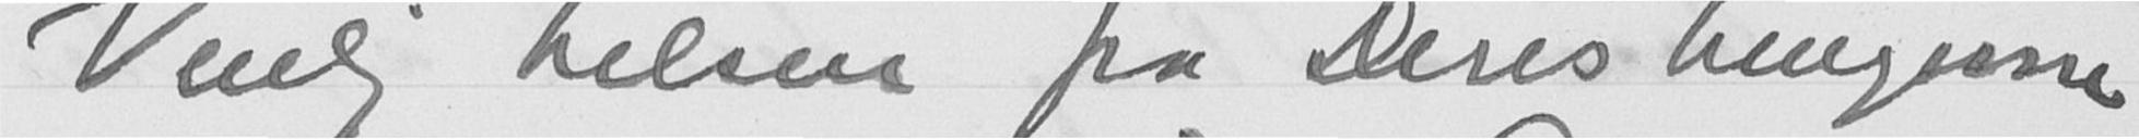Venlig hilsen fra Deres hengivne
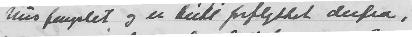hus fengslet og er blitt forflyttet derfra,
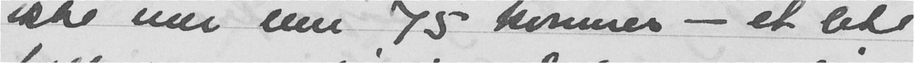ikke mer enn 75 kvinner - et lite
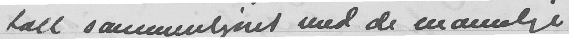tall sammenlignet med de mannlige
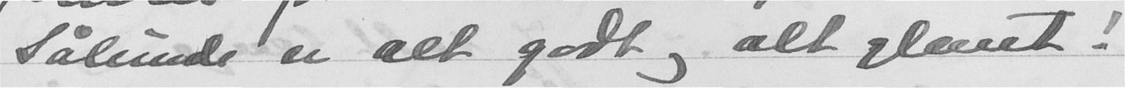Sålunde er det godt og alt glemt!
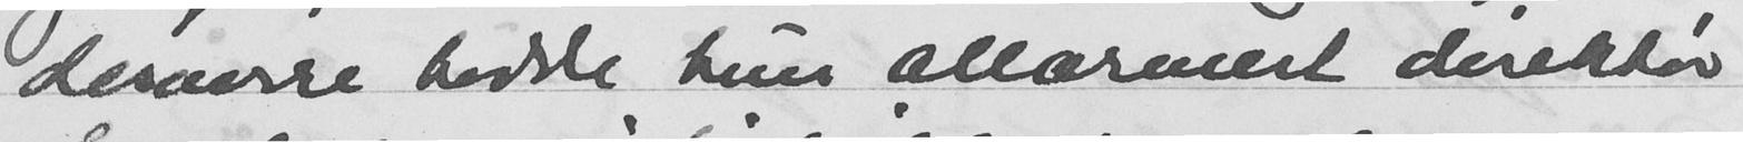desverre hadde hun allarmert direktør
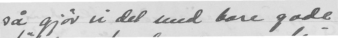så gjør vi det med bare gode
